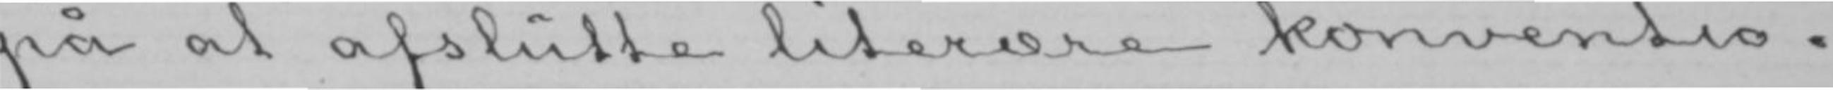på at afslutte literære konventio-
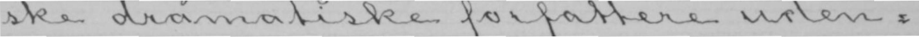ske dramatiske forfattere uden-
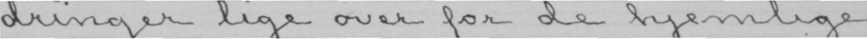dringer lige over for de hjemlige
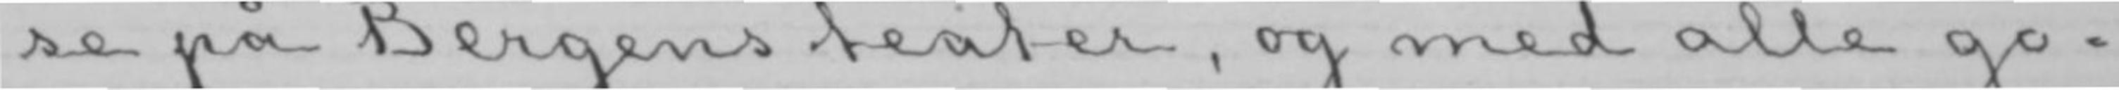se på Bergens teater, og med alle go-
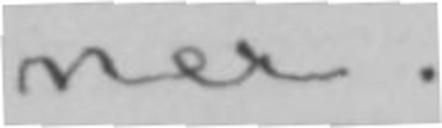ner.
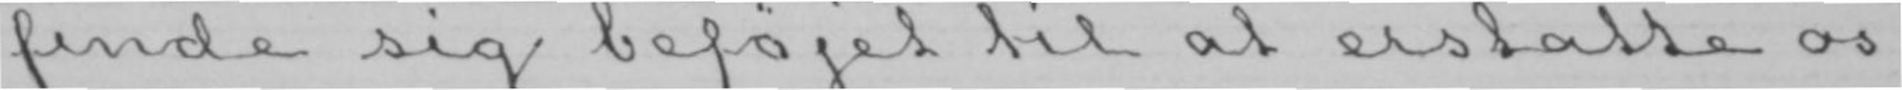finde sig beføjet til at erstatte os
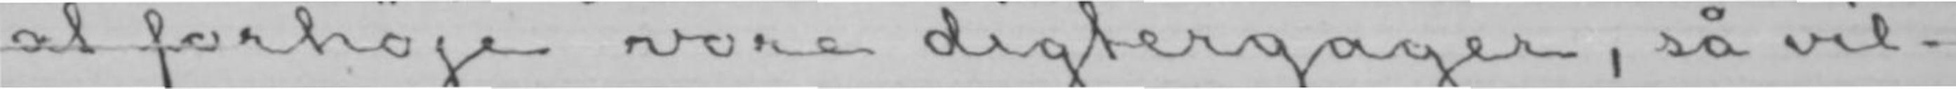at forhøje vore digtergager, så vil-
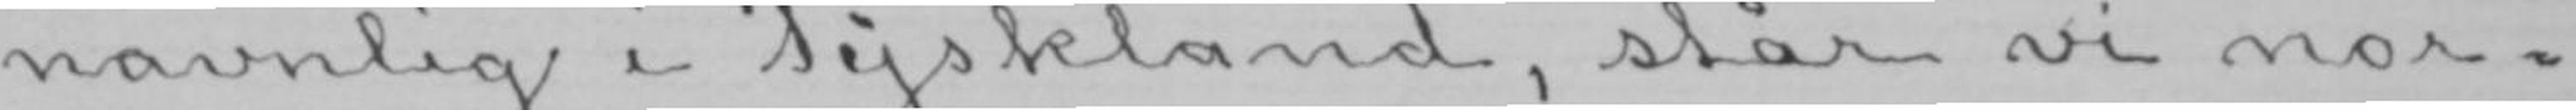navnlig i Tyskland, står de nor-
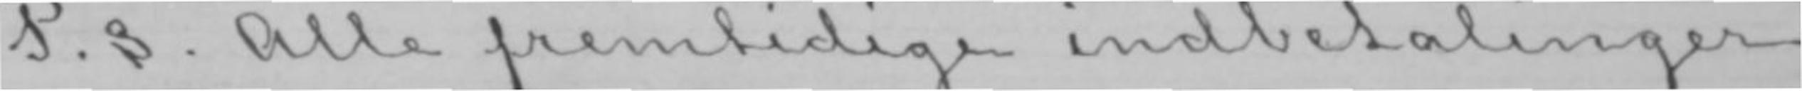P. s. Alle fremtidige indbetalinger
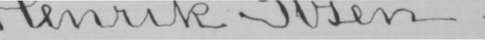Henrik Ibsen.
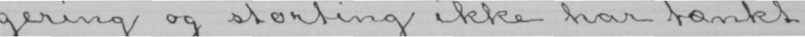gering og storting ikke har tænkt
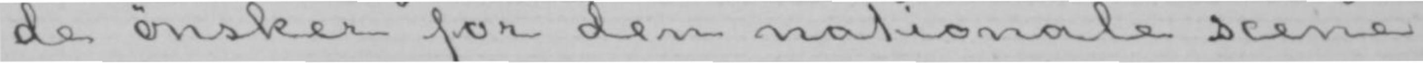de ønsker for den nationale scene
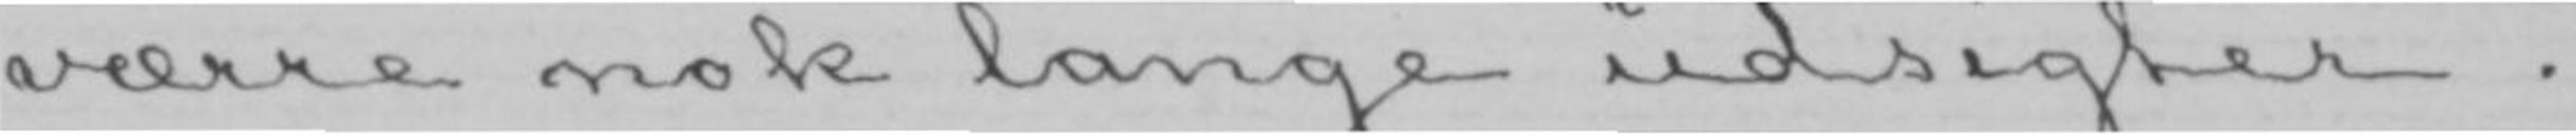værre nok lange udsigter.
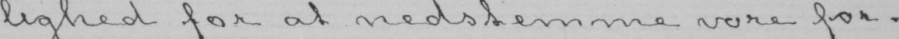lighed for at nedstemme vore for-
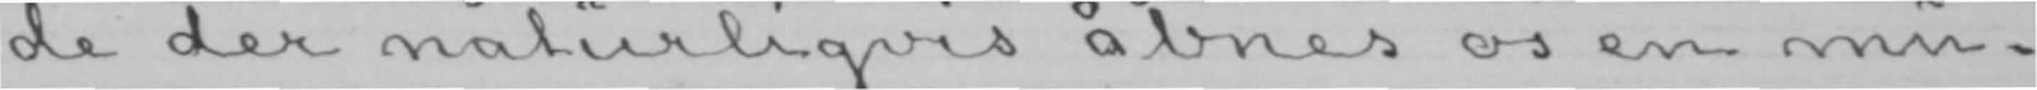de der naturligvis åbnes os en mu-
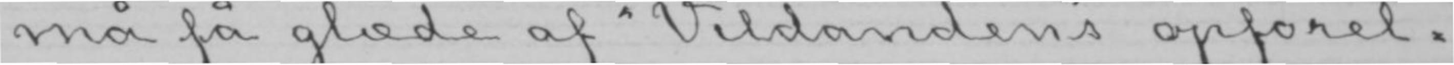må få glæde af «Vildanden»s opførel-
